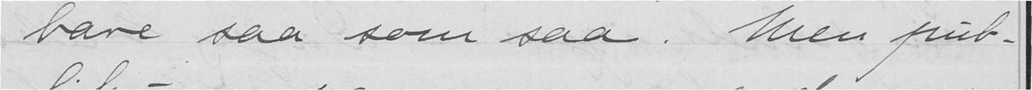bare saa som saa. Men pub-
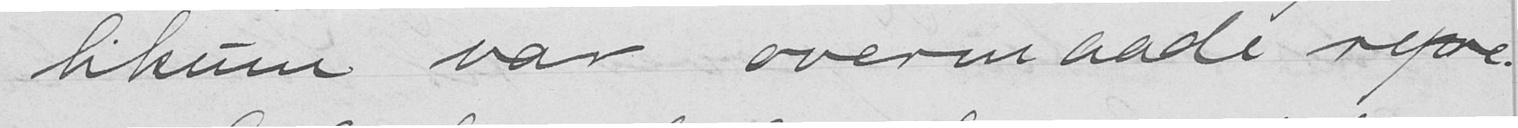likum var overmaade repre-
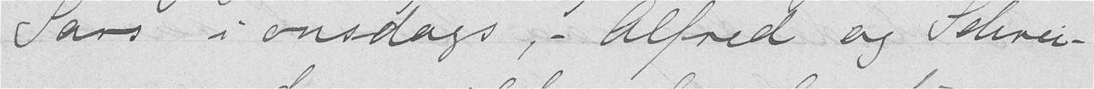Sars i onsdags, - Alfred og Schrei-
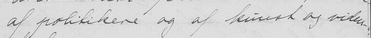af politikere og af kunst og viden-
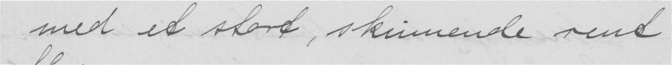med et stort, skinnende rent
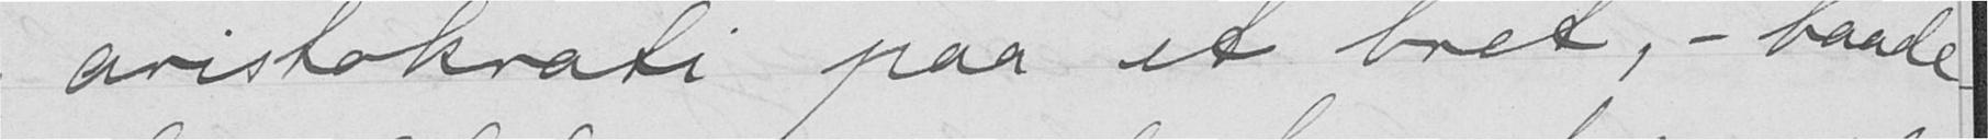aristokrati paa et bret, - baade
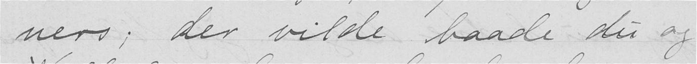ners; der vilde baade du og
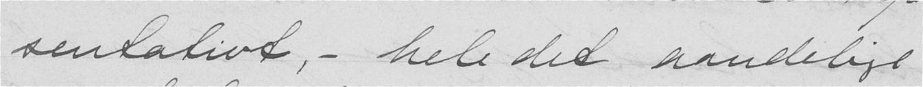sentativt, - hele det aandelige
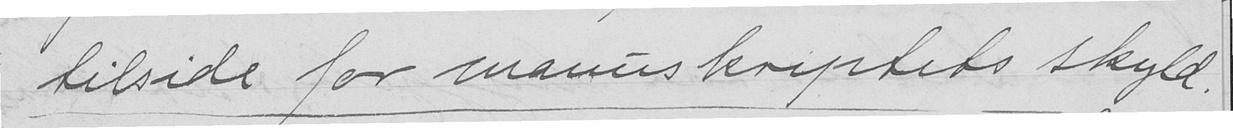tilside for manuskriptets skyld.
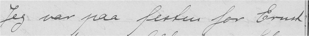Jeg var paa festen for Ernst
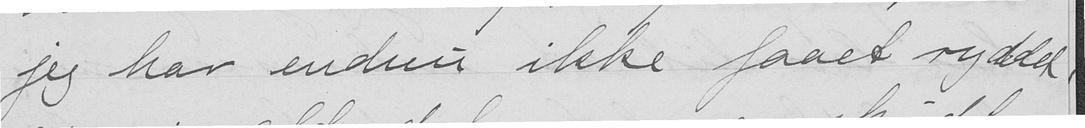jeg har endnu ikke faaet ryddet
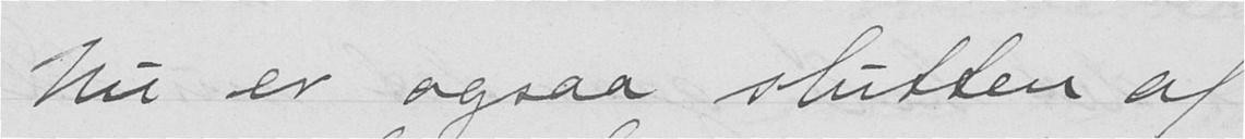Nu er ogsaa slutten af
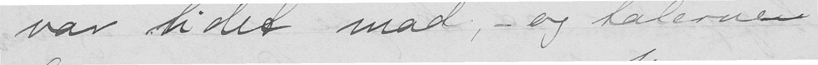var lidet mad, - og talerne
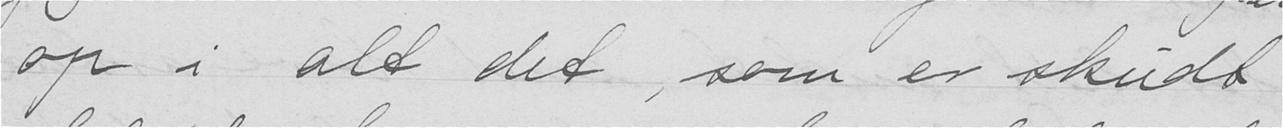op i alt det, som er skudt
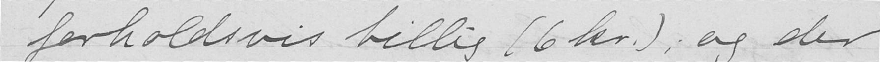forholdsvis billig (6 kr.); og der
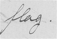flag.
